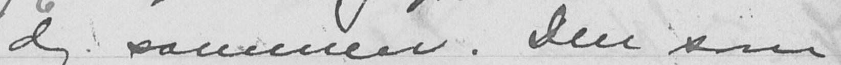dag sammen. Den som
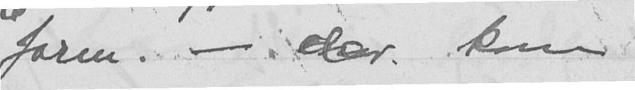form. - der kom
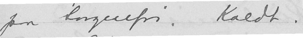paa Sorgenfri. Koldt.
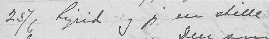25/6 Sigrid og jeg en stille
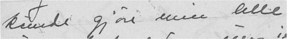kunde gjøre min lille
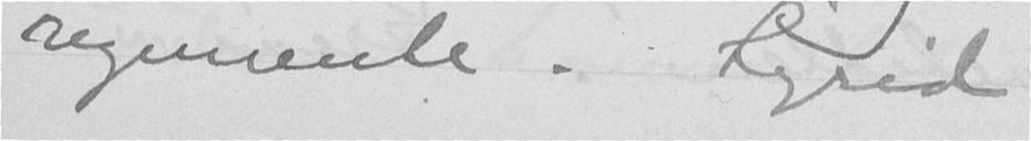regimente. Sigrid
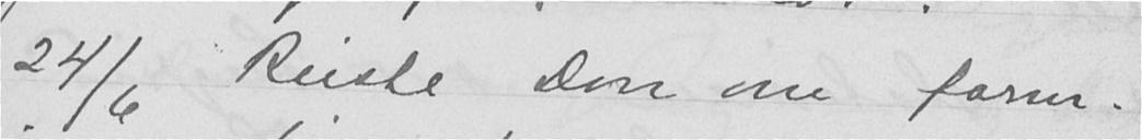24/6 Reiste Don om form.
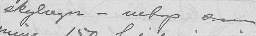skylregn - netop som
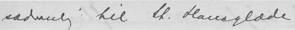sædvanlig til St. Hansglæde
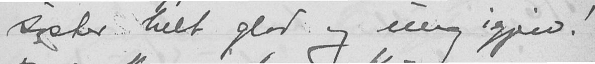søster helt glad og ung igjen!
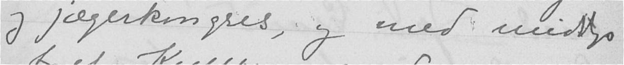og jægerkongres, og med middags
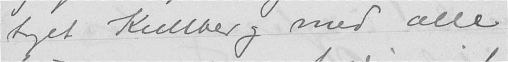toget Kullberg med alle
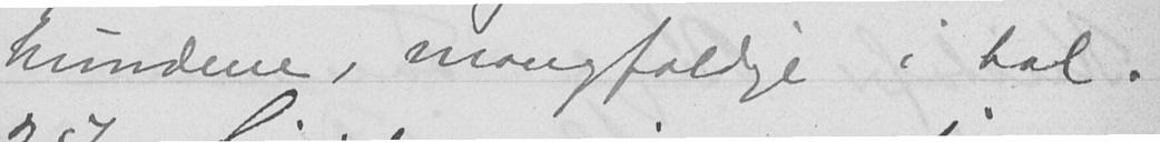hundene, mangfoldige i tal.
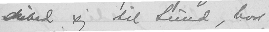skibed sig til Sund, hvor
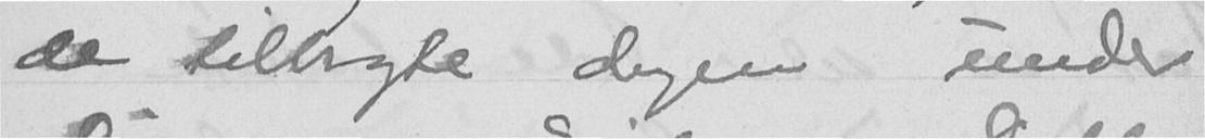de tilbragte dagen under
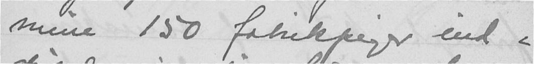mine 150 fabrikpiger ind-
